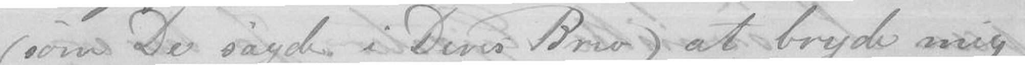(som De sagde i Deres Brev) at bryde mig
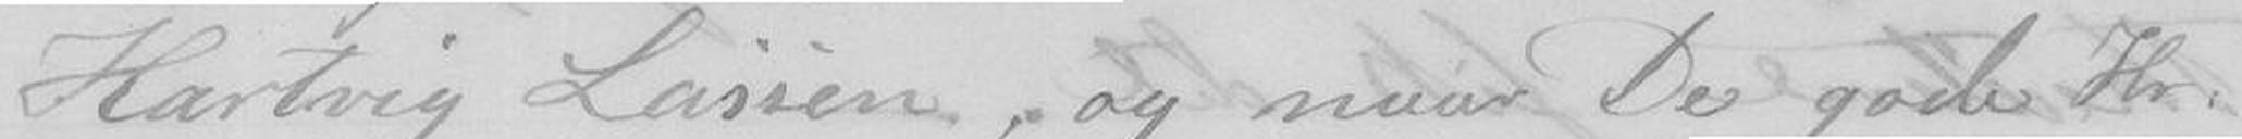Hartvig Lassen, og naar De gode Hr.
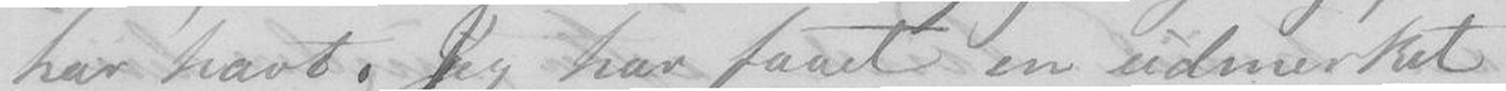har havt. Jeg har faaet en udmerket
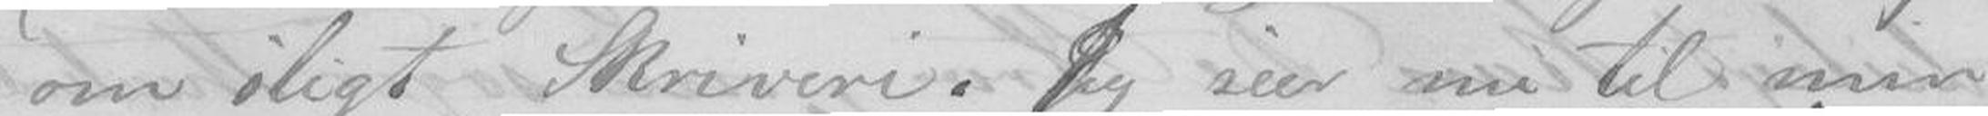om sligt Skriveri. Jeg seer nu til min
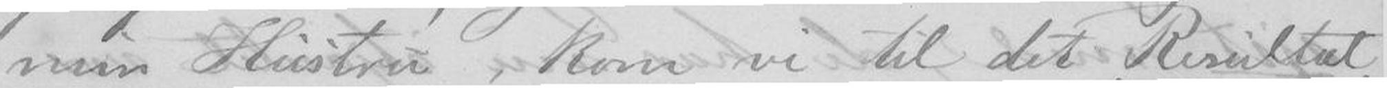min Hustru, kom vi til det Resultat,
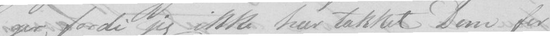ger, fordi jeg ikke har takket Dem for
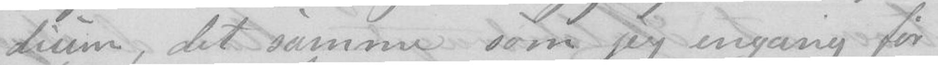dium, det samme som jeg engang før
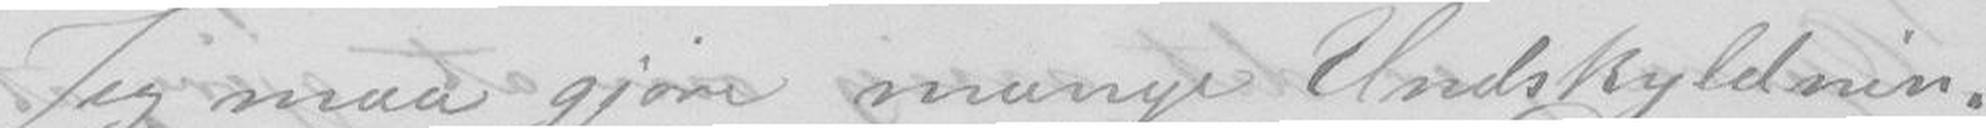Jeg maa gjøre mange Undskyldnin-
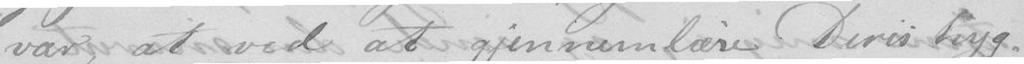var, at ved at gjennemlæse Deres hyg-
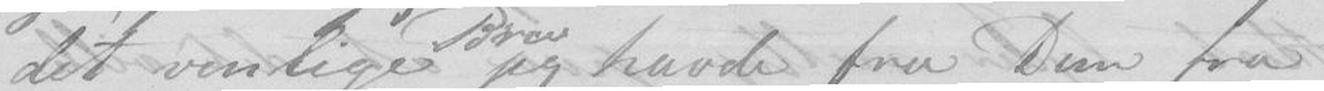det venlige jeg havde fra Dem fra
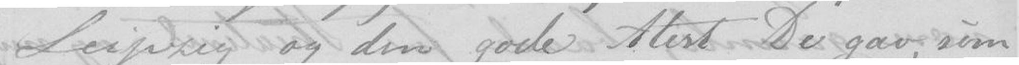Leipzig og den gode Atest De gav, som
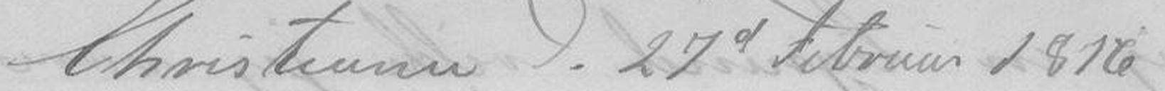Christiania d. 27d Februar 1876
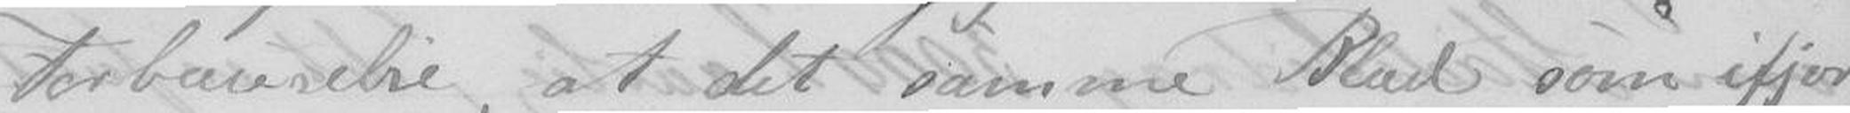Forbauselse, at det samme Blad som ifjor
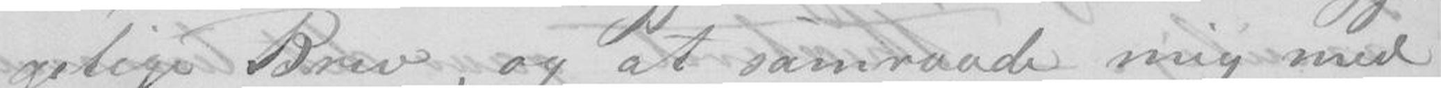gelige Brev, og at samraade mig med
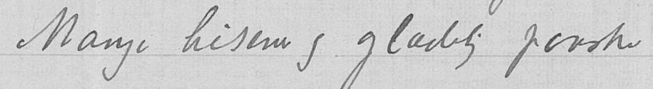Mange hilsener og glædelig paaske
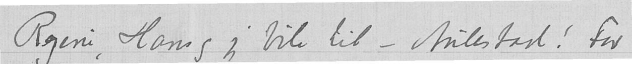Regine, Hans og jeg bile til – Aulestad! For
